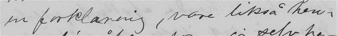en forklaring, være likså hen-
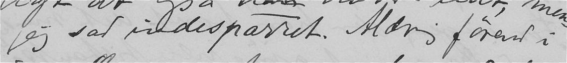jeg sad indespærret. Aldrig førend i
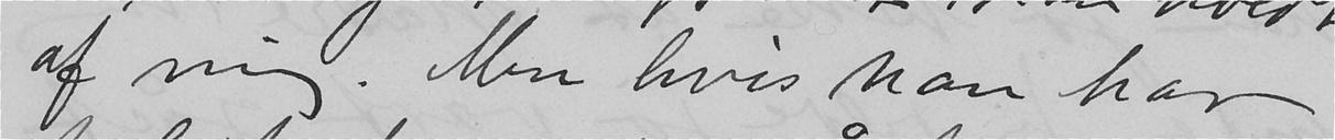af mig. Men hvis han har
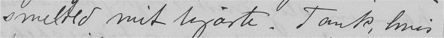smelted mit hjærte. Tænk, hvis
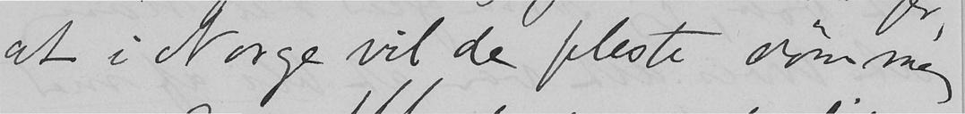at i Norge vil de fleste dømme
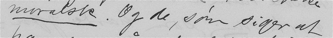moralsk. Og de, som siger at
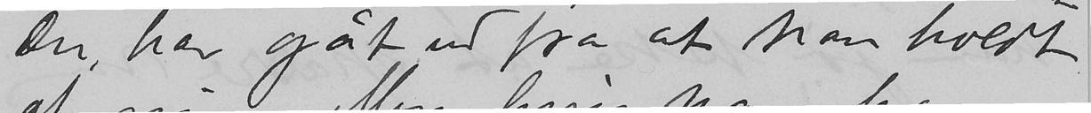Du, har gåt ud fra at han holdt
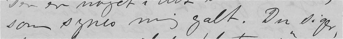som synes mig galt. Du siger,
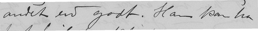andet end godt. Han kan ha
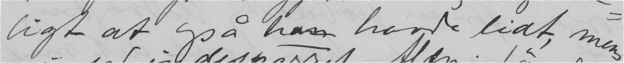ligt at også han havde lidt, mens
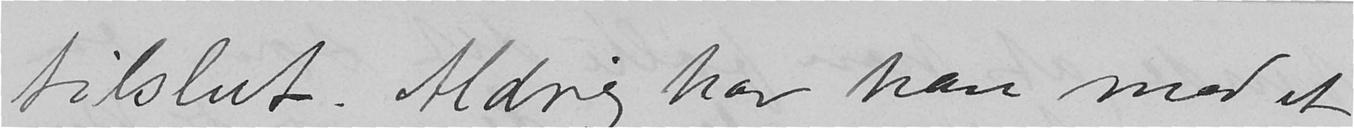tilslut. Aldrig har han med et
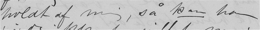holdt af mig, så kan han
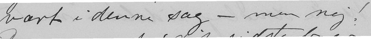vært i denne sag - men nej!
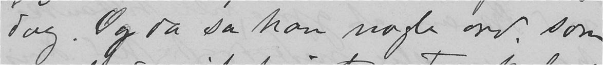dag. Og da sa han nogle ord, som
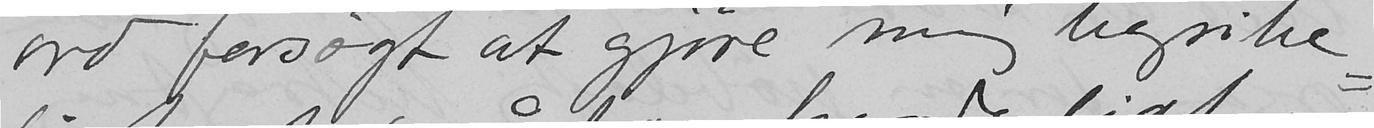ord forsøgt at gjøre mig begribe-
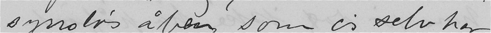synsløs åben, som jeg selv har
